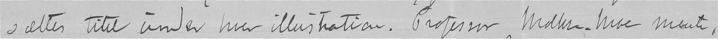sættes titel under hver illustration. Professor Moltke Moe mente,
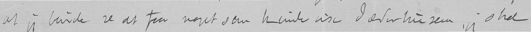at jeg burde se at faa noget som kunde vise Jæderhusene, jeg skal
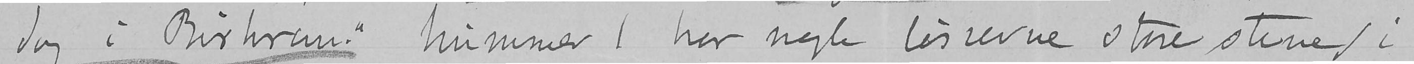dag i Birkrem." Nummer I har nogle løsrevne store stene i
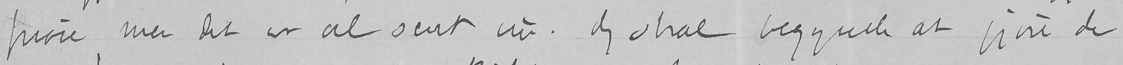prøve, men det er vel sent nu. Jeg skal begynde at gjøre de
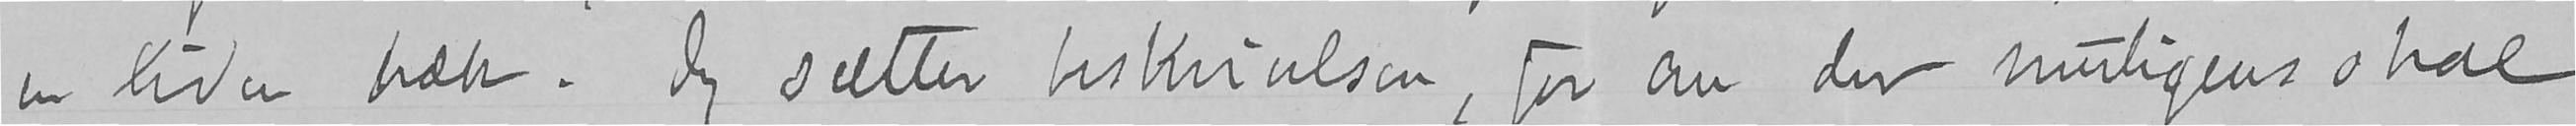en liden gæter. Jeg sætter beskrivelsen, for om der muligens skal
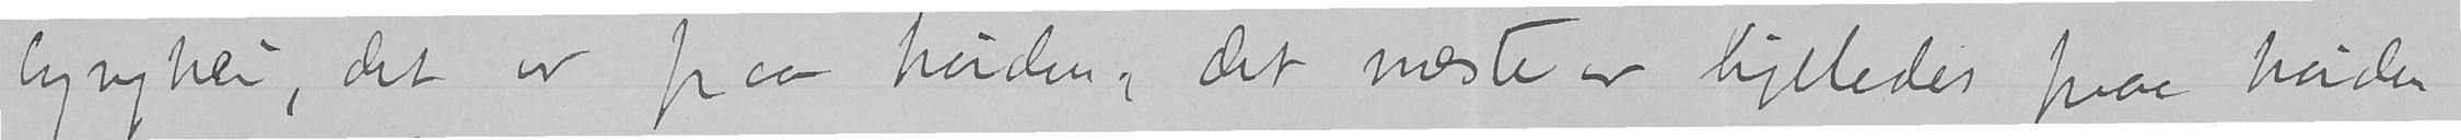lynghei, det er paa høiden, det næste er ligeledes paa høiden
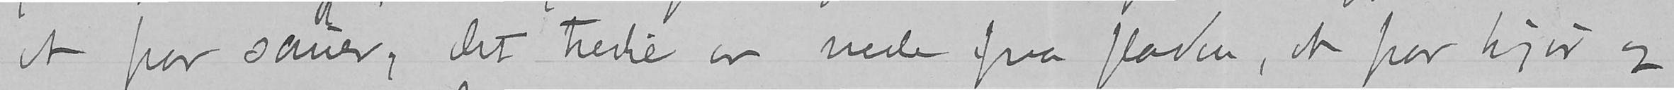et par sauer, det tredie er nede paa fladen, et par kyr og
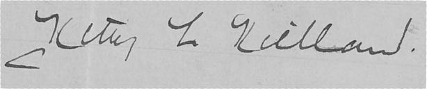Kitty L. Kielland.
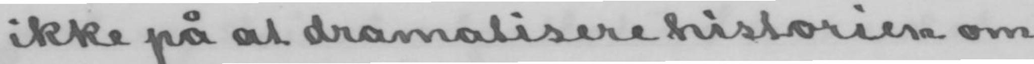ikke på at dramatisere historien om
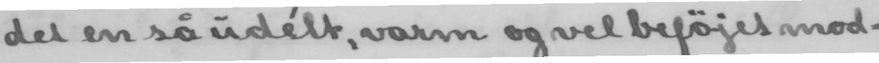det en så udélt, varm og vel beføjet mod-
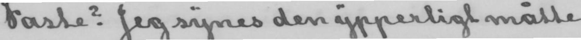Faste? Jeg synes den ypperligt måtte
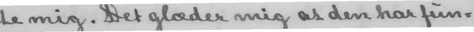te mig. Det glæder mig at den har fun-
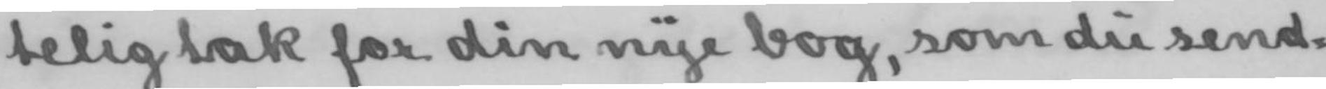telig tak for din nye bog, som du send-
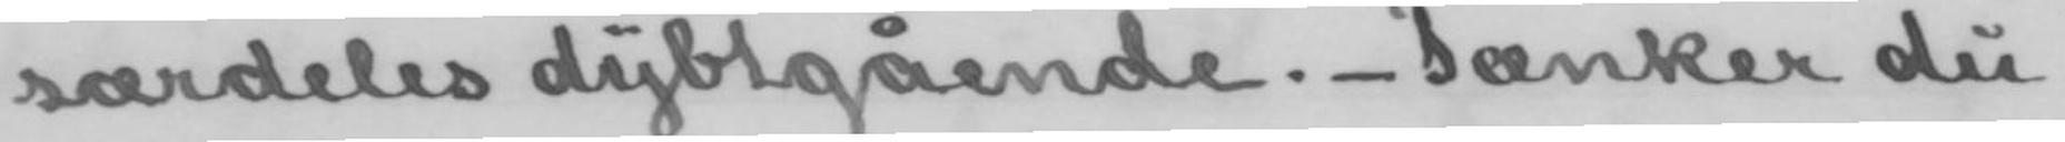særdeles dybtgående.- Tænker du
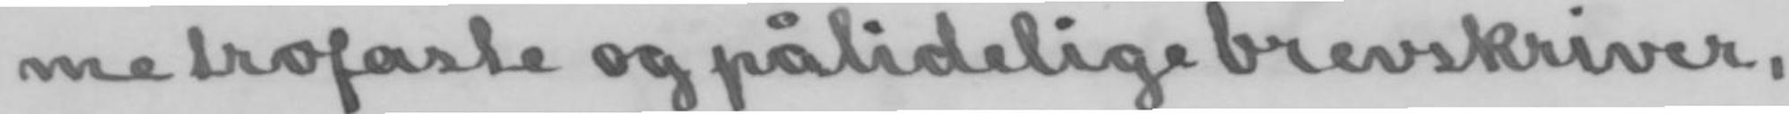me trofaste og pålidelige brevskriver,
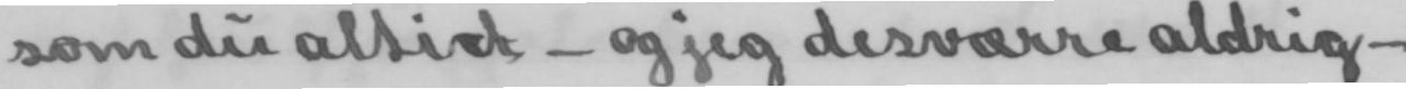som du altid- og jeg desværre aldrig-
